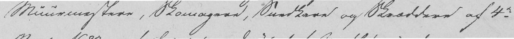Muurmestere, Skomagere, Snedkere og Skræddere af 4de
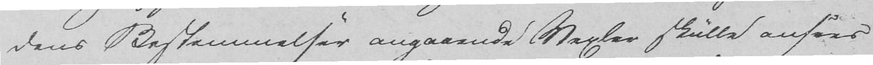dens Bestemmelser angaaende Vexler skulle ansees
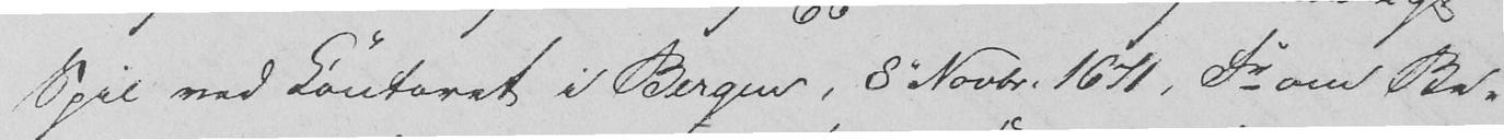Spil ved Contoret i Bergen, 8 Novbr. 1671, Fr om Be-
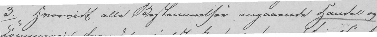3. Hvorvidt alle Bestemmelser angaaende Handel og
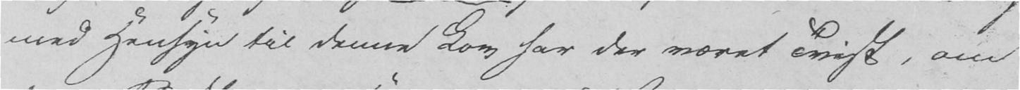med Hensyn til denne Lov har der været Tvist, om
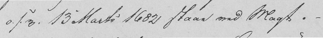o. s. v. 13 Marts 1682 staae ved Magt. -
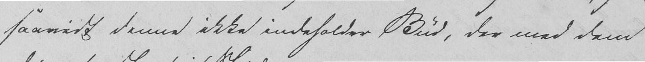saavidt denne ikke indeholder Bud, der med dem
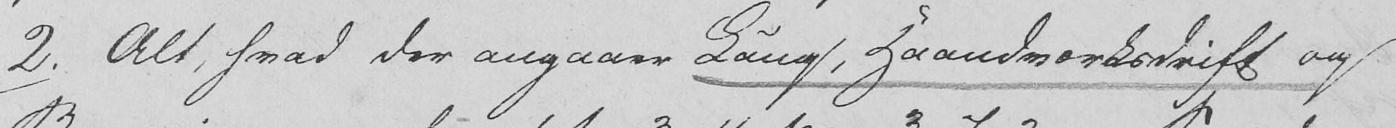2. Alt hvad der angaaer Laug, Haandværksdrift og
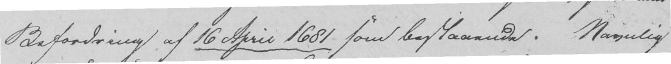Befordring af 16 April 1681 som bestaaende. Navnlig
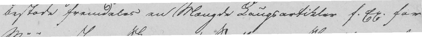bestode fremdeles en Mængde Laugsartikler f. Ex. for
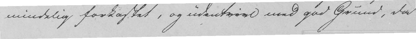mindelig forkastet, og udentvivl med god Grund, da
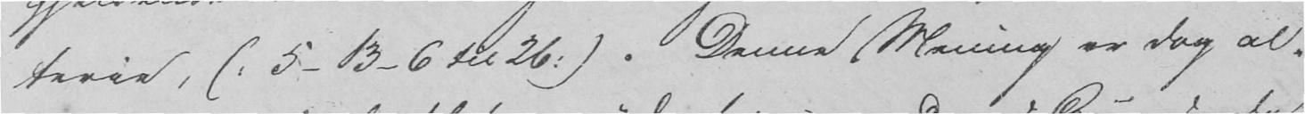terie, (: 5-13-6 til 26 :). Denne Mening er dog al-
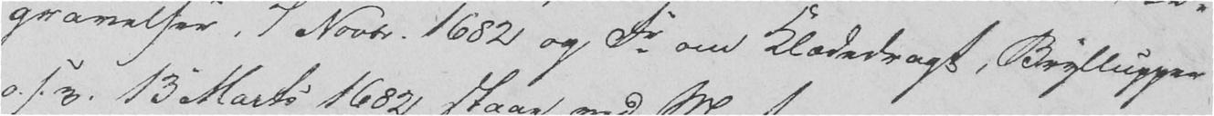gravelser, 7 Novbr. 1682 og Fr om Klædedragt, Bryllupper
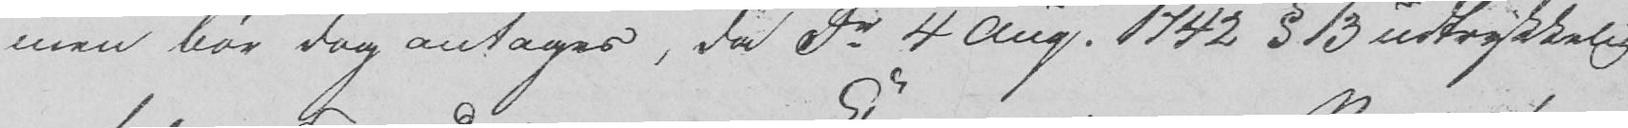men bør dog antages, da Fr 4 Aug. 1742 § 13 udtrykkelig
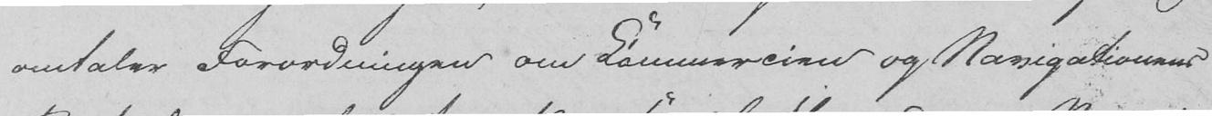omtaler Forordningen om Commercien og Navigationens
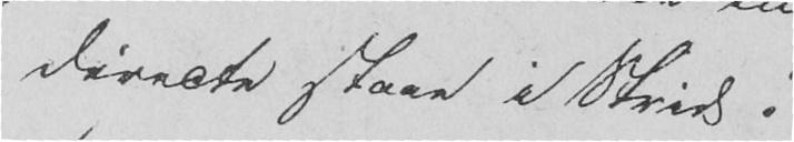directe staar i Strid.
